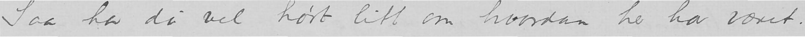Saa har du vel hørt litt om hvordan her har været.
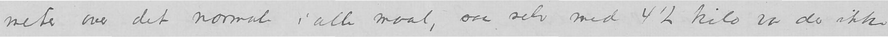meter over det normale i alle maal, saa selv med 4 ½ kilo var der ikke
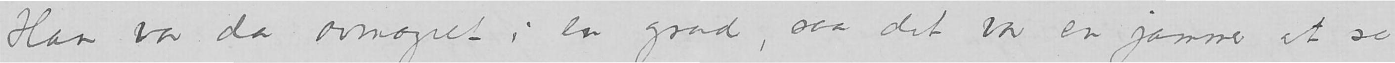Han var da avmagret i en grad, saa det var en jammer at se
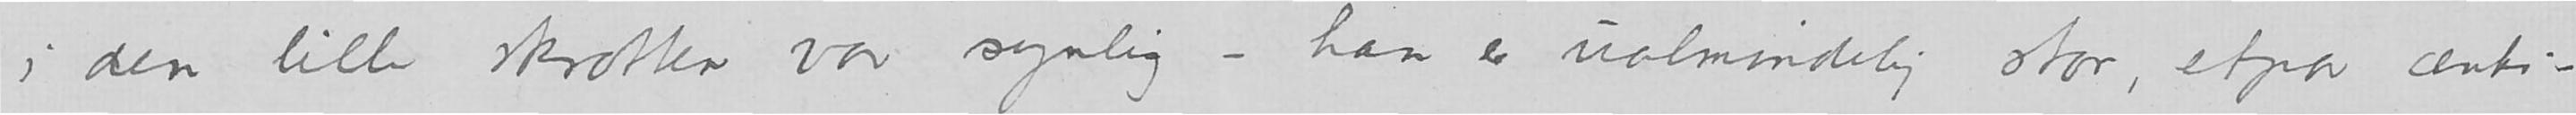i den lille skrotten var synlig – han er ualmindelig stor,m etpar centi-
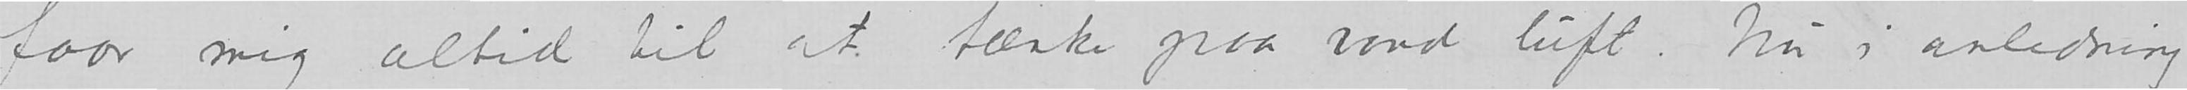faar mig altid til at tænke paa vond luft. Nu i anledning
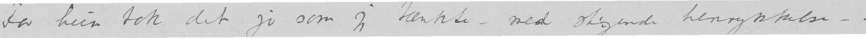For hun tok det jo som jeg tænkte – med stigende henrykkelse –.
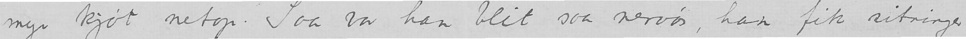mye kjøt netop. Saa var han blit saa nervøs, han fik sitringer
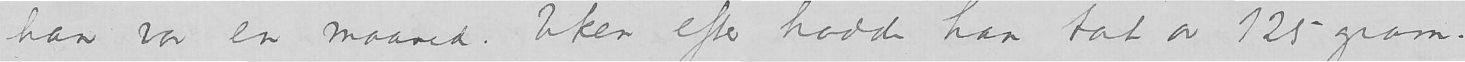han var en maaned. Uken efter hadde han tat av 125 gram.
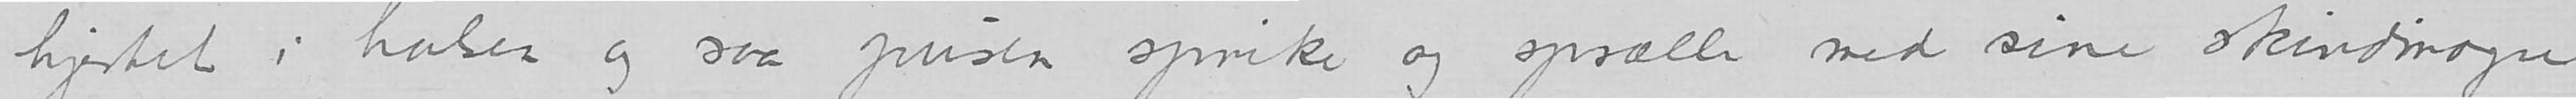hjertet i halsen og saa pusen sprike og sprælle med sine skindmagre
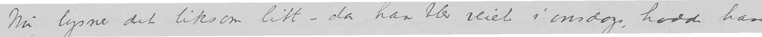Nu lysner det liksom litt – da han blev veiet i onsdags hadde han
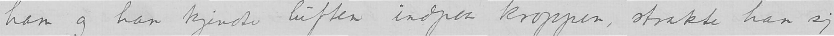ham og han kjendte luften indpaa kroppen, straktek han sig
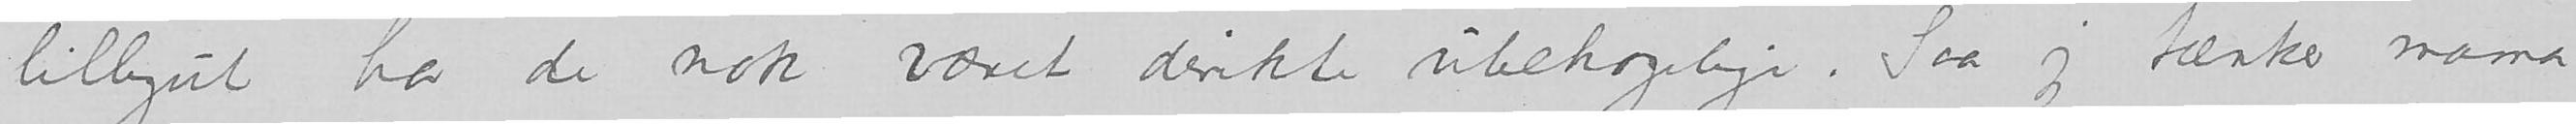lillegut har de nok været direkte ubehagelige. Saa jeg tænker mama
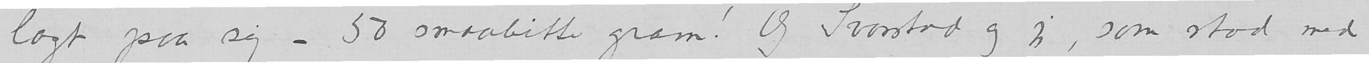lagt paa sig – 50 smaabitte gram! Og Svarstad og jeg, som stod med
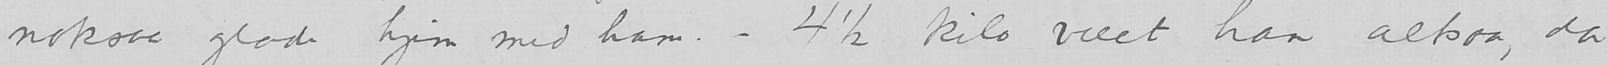noksaa glade hjem med ham. – 4 ½ kilo veiet han altsaa, da
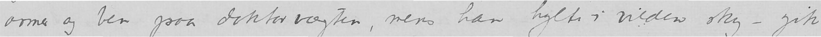armer og ben paa doktorvægten, mens han hylte i vilden sky – gik
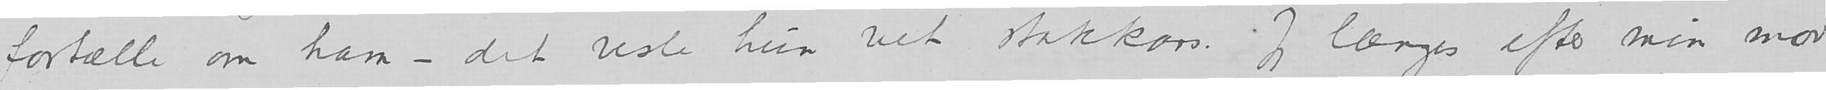fortælle om ham – det vesle hun vetd stakkars. Jeg længes efter min mor
